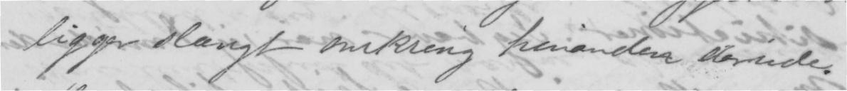ligger slængt omkring hinanden derude.
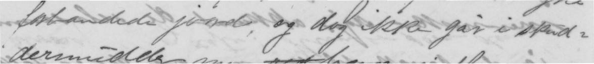forbandede jord og dog ikke går i skud-
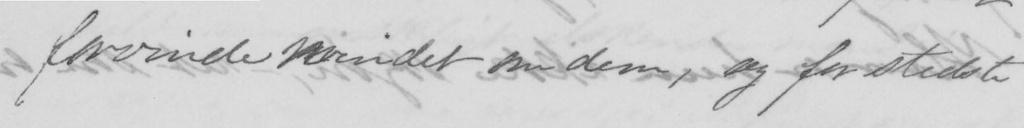forvinde mindet om dem, og for stedse
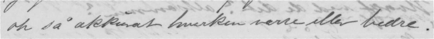oh så akkurat hverken verre eller bedre.
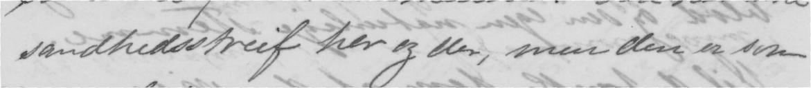sandhedsstreif her og der, men den er som
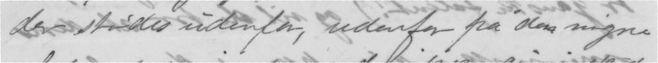der stødes udenfor, udenfor på den nøgne
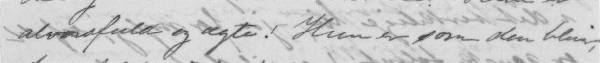alvorsfuld og ægte! Hun er som den bliver,
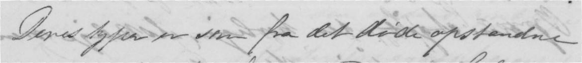Deres typer er som fra det døde opstandne
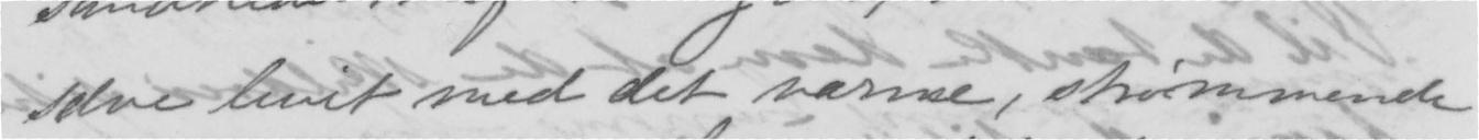selve livet med det varme, strømmende
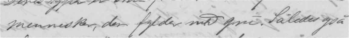mennesker, der fyldes med gru. Således også
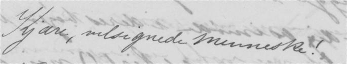Kjære, velsignede menneske!
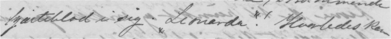hjærteblod i sig. Leonarda! Hvorledes kan
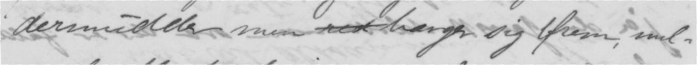dermudder, men red bærger sig frem, mel-
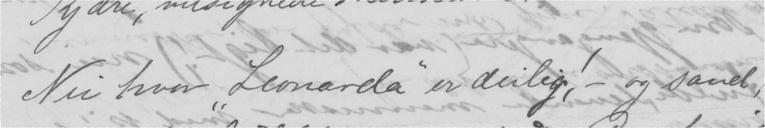Nei hvor "Leonarda" er deilig! - og sand,
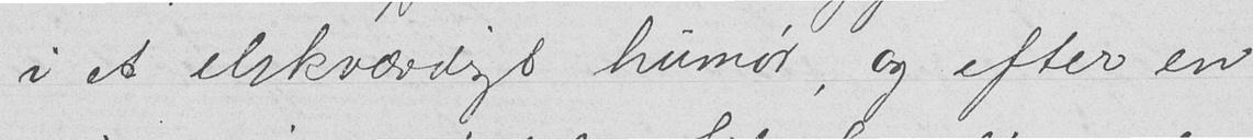i et elskværdigt humør, og efter en
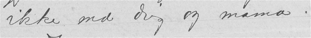ikke med dig og mama.
